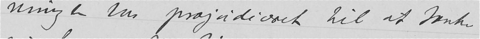ningen var prejudiceret til at tænke
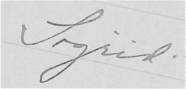Sigrid.
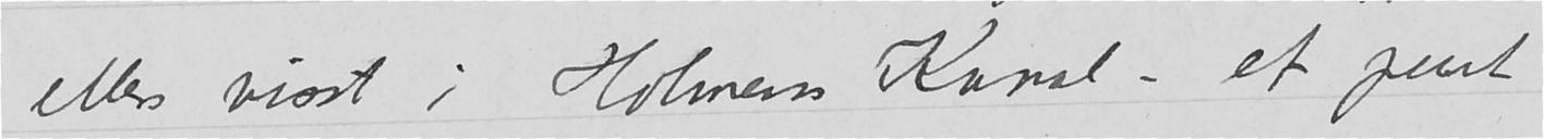ellers visst i Holmens Kanal – et pent
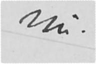nu.
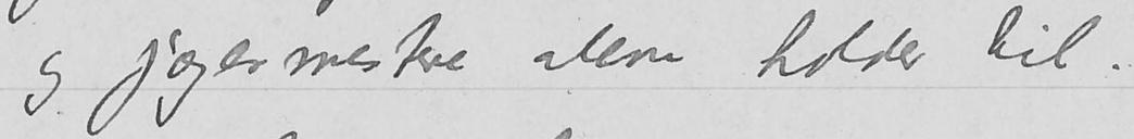og jægermestere alene holder til.
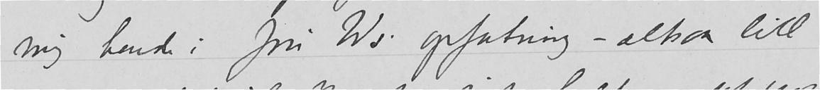mig hende i fru Ws. opfatning – altsaa litt
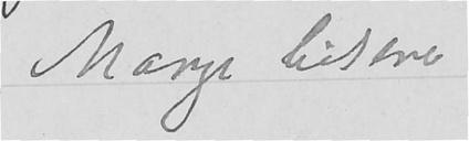Mange hilsener
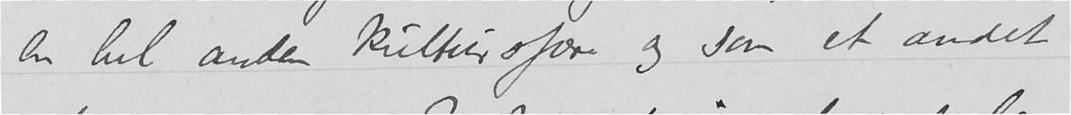en hel anden kultursfære og som et andet
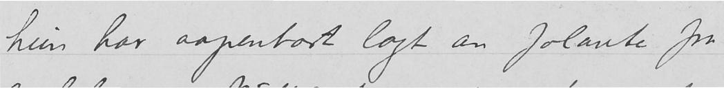hun har aapenbart lagt an Jolante fra
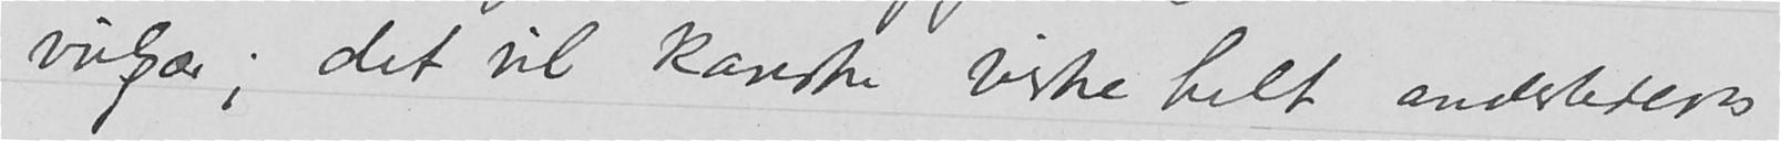vulgær; det vil kanske virke helt anderledens
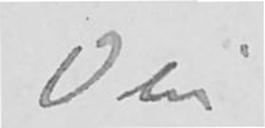Din
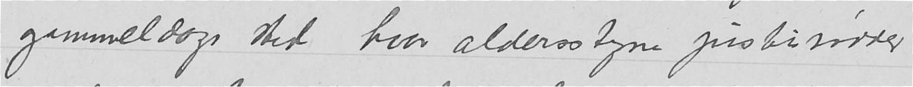gammeldags sted hvor aldersstegne justisriddere
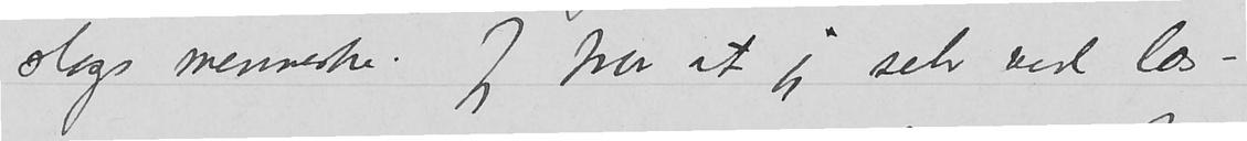slags menneske. Jeg tror at jeg selv ved læs –
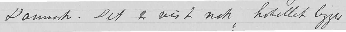Danmark. Det er vist nok hotellet ligger
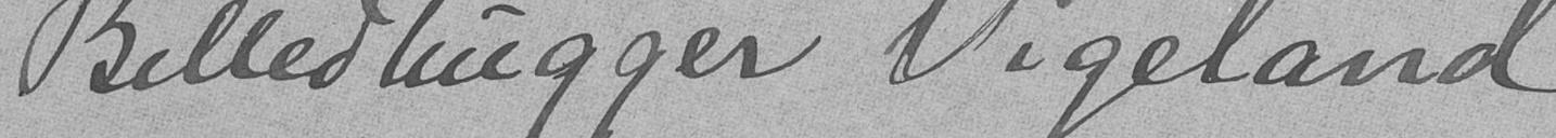Billedhugger Vigeland
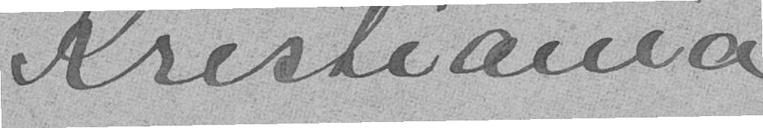Kristiania
